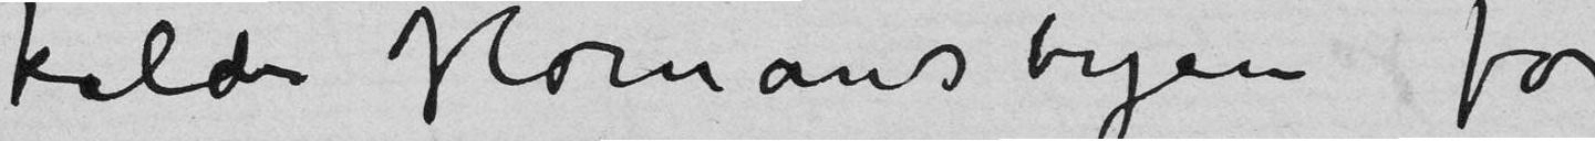kalder Homansbyen for
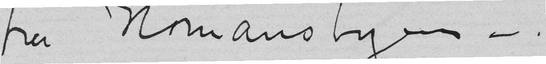fra Homansbyen –
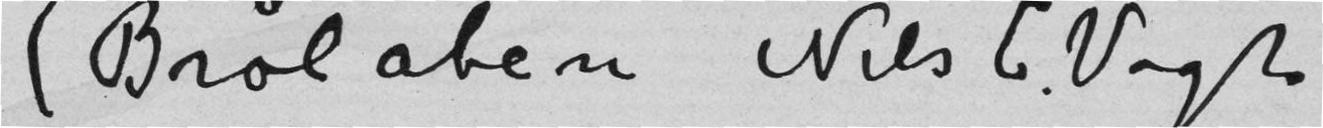(Brølaben Nils C. Vogt
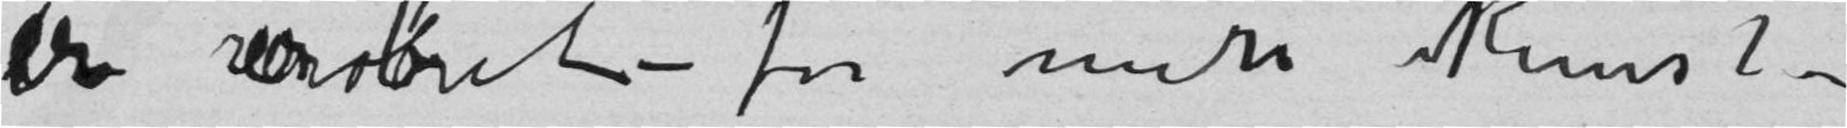er erobret for min Kunst –
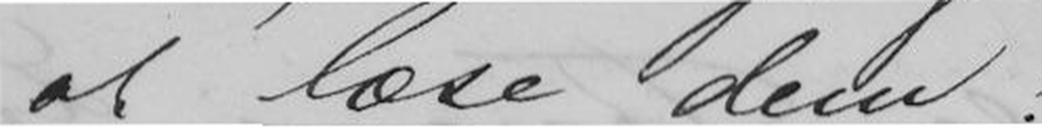at læse dem!
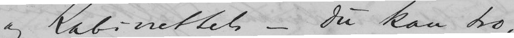og Kabinettet - du kan tro,
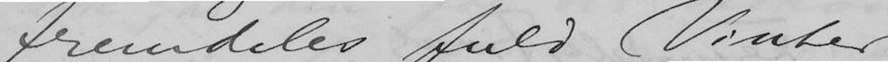fremdeles fuld Vinter
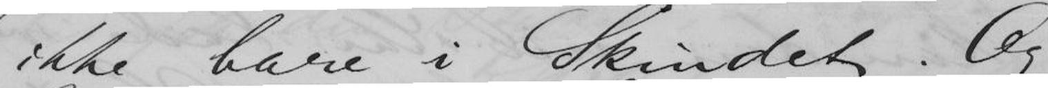ikke bare i Skindet. Og
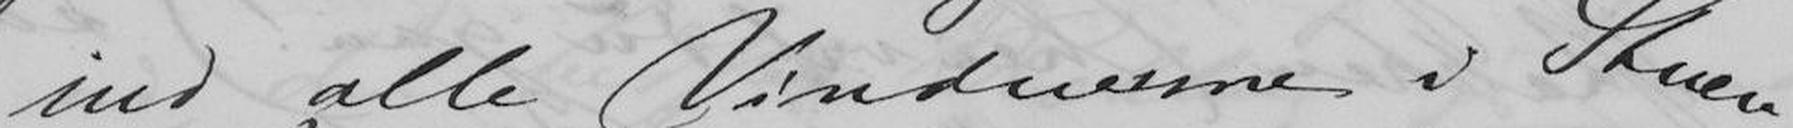ind alle Vinduerne i Stuen
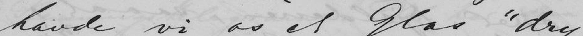havde vi os et Glas "dry"
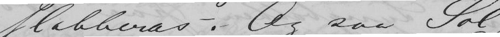slabberas -. Og saa Sol
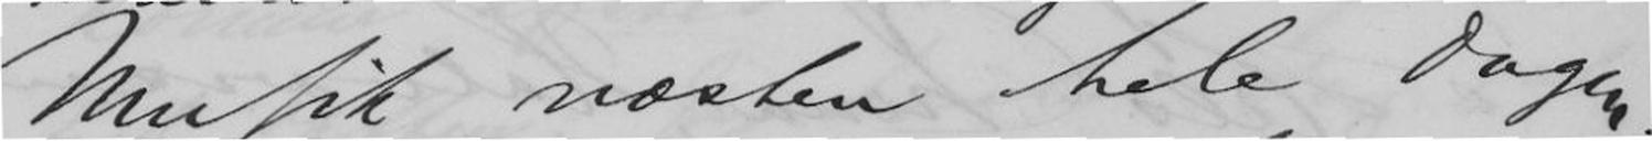Musik næsten hele Dagen.
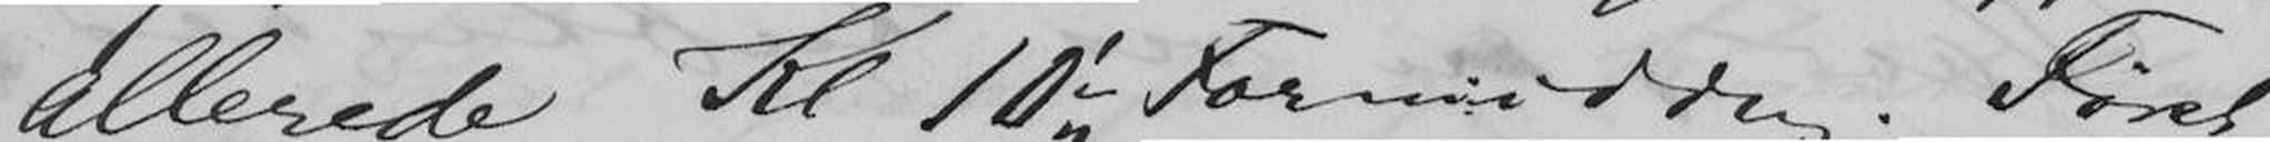allerede Kl 10 ½ Formiddag. Først
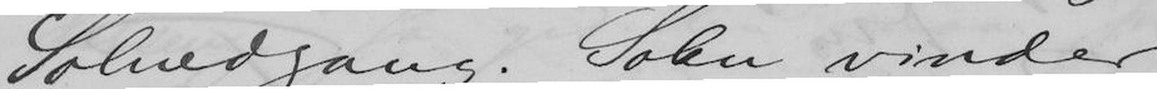Solnedgang. Solen vinder
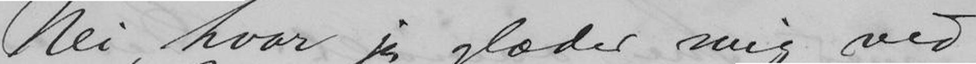Nei, hvor jeg glæder mig ved
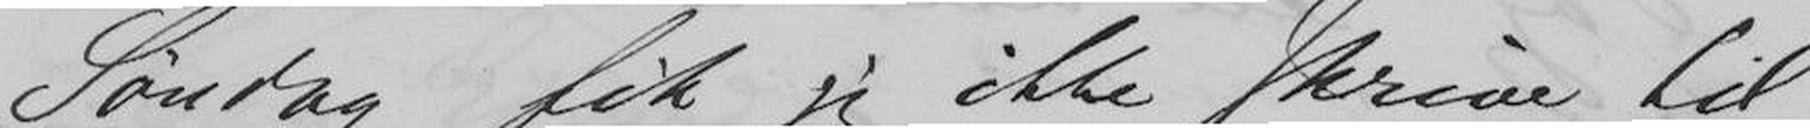Søndag fik jeg ikke skrive til
